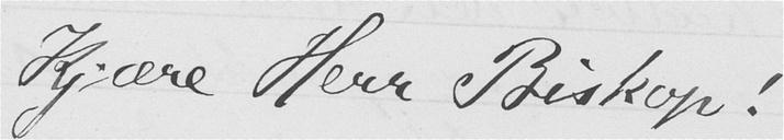Kjære Herr Biskop!
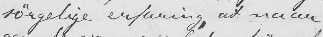sørgelige erfaring, at naar
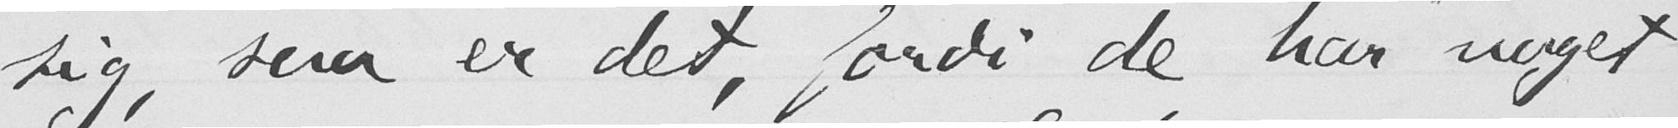sig, saa er det, fordi de har noget
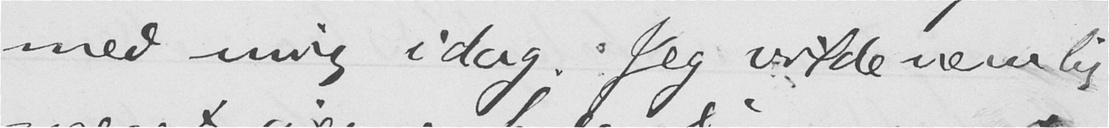med mig i dag. Jeg vilde nemlig
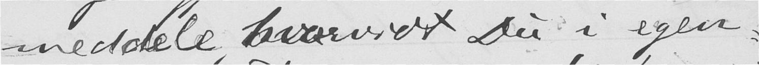meddele, hvorvidt Du i egen-
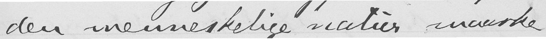den menneskelige natur maaske
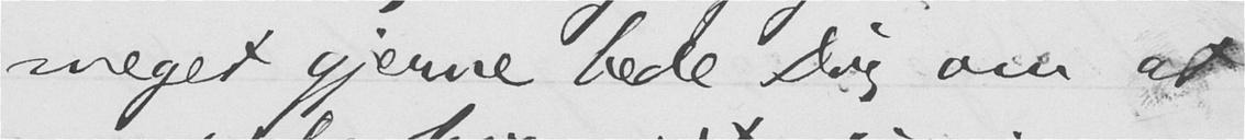meget gjerne bede Dig om at
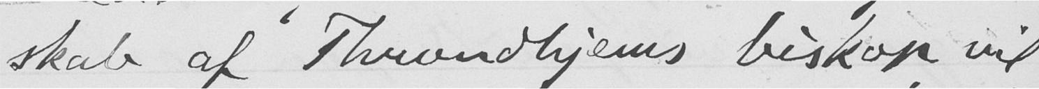skab af Throndhjems biskop vil
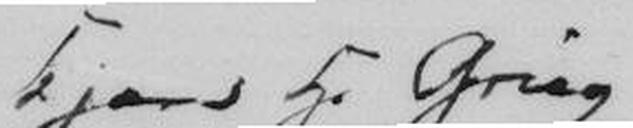Kjære Hr Grieg
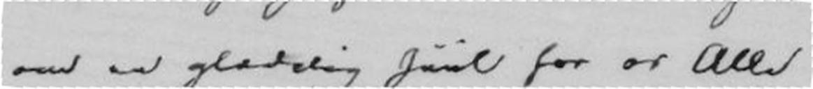om en glædelig Juul for er Alle
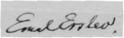Emil Erslev.
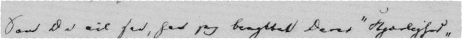Som De vil se, har jeg benytted Deres "Kjærlighed"
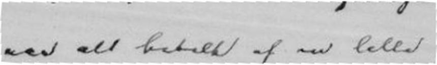var alt betalt af en lalla
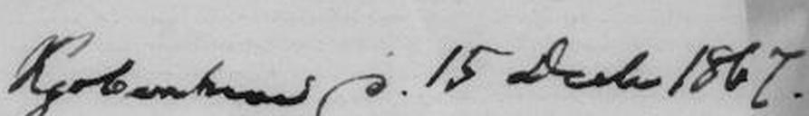Kjøbenhavn d. 15 Decbr 1867.
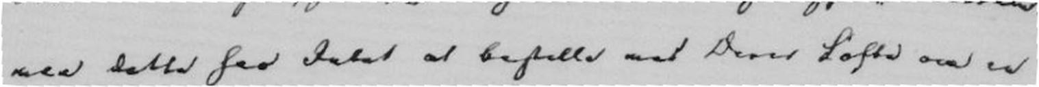men dette har Intet at bestille ved Deres Løfte om en
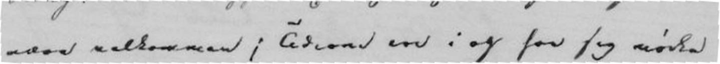være velkommen; Åd... ere i og for sig mørka
